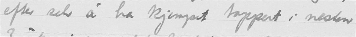efter selv å ha kjempet tappert i nesten
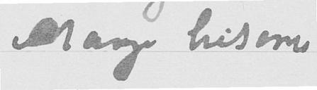Mange hilsener
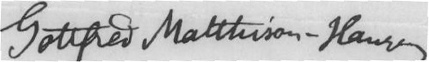Gottfred Matthison-Hansen.
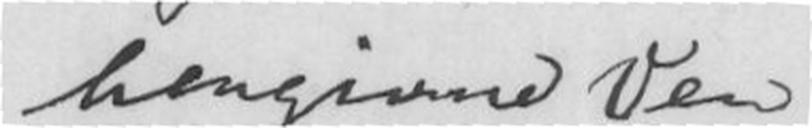hengivne Ven
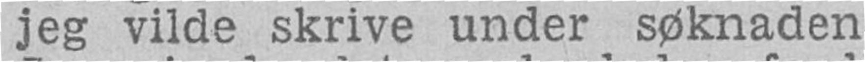jeg vilde skrive under søknaden.
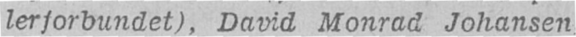lerforbundet), David Monrad Johansen,
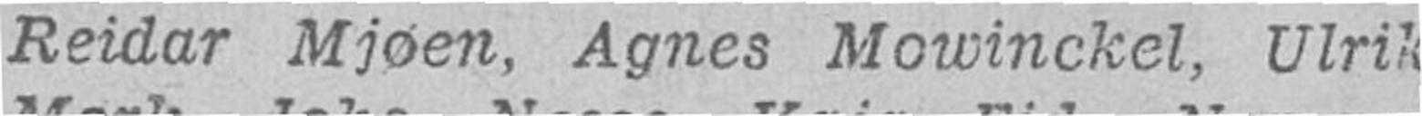Reidar Mjøen, Agnes Mowinckel, Ulrik
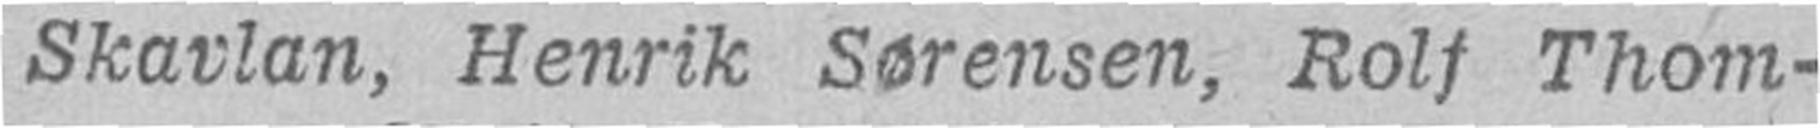Skavlan, Henrik Sørensen, Rolf Thom-
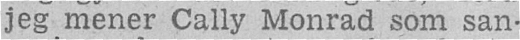jeg mener Cally Monrad som san-
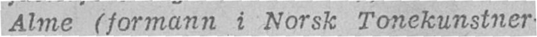Alme (formann i Norsk Tonekunstner-
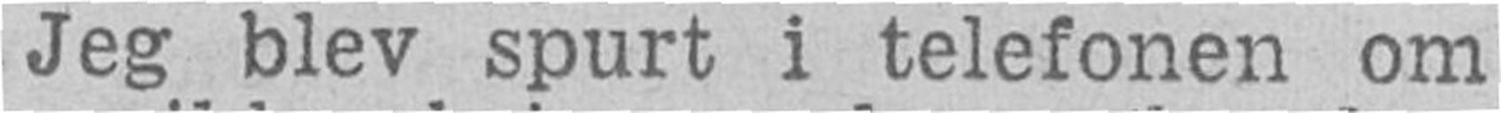Jeg blev spurt i telefonen om
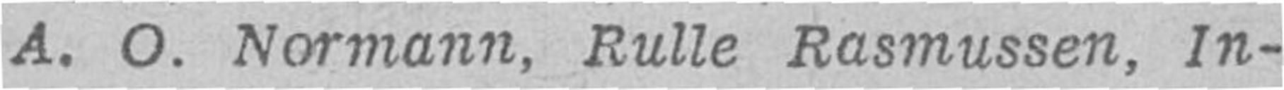A. O. Normann, Rulle Rasmussen, In-
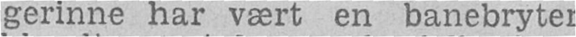gerinne har vært en banebryter
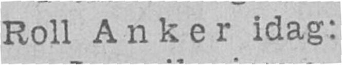Roll Anker idag:
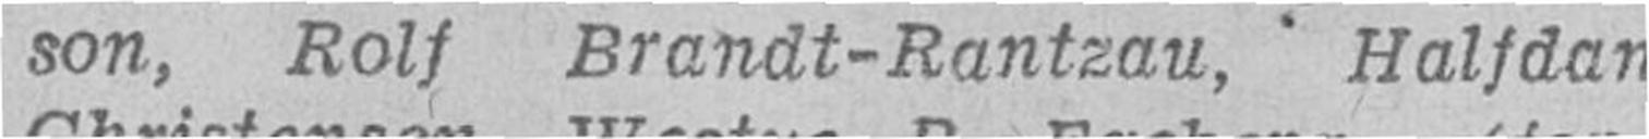son, Rolf Brandt-Rantzau, Halfdan
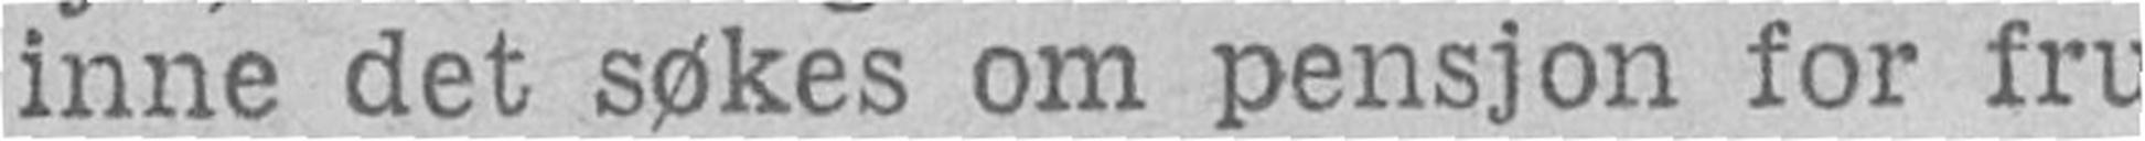inne det søkes om pensjon for fru
